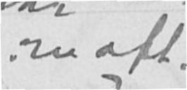om aft.
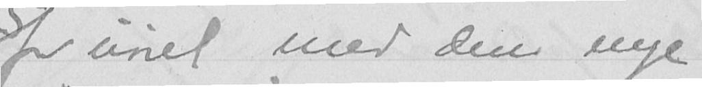fornøiet med den nye
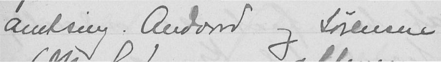amtsing. Andvord og Sørensen
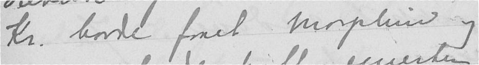Kr. havde faaet morphin og
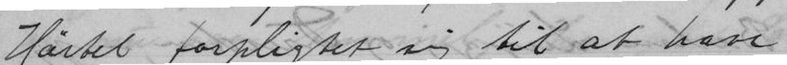Härtel forpliktet sig til at have
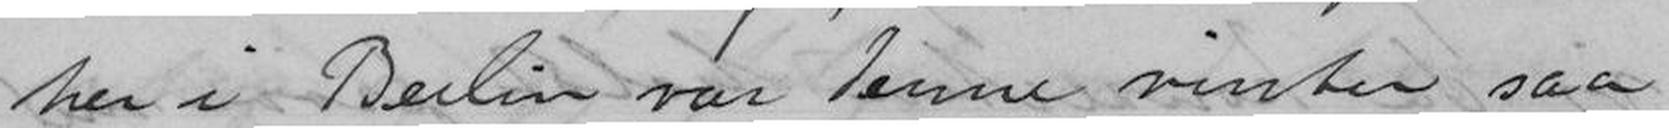her i Berlin var denne vinter saa
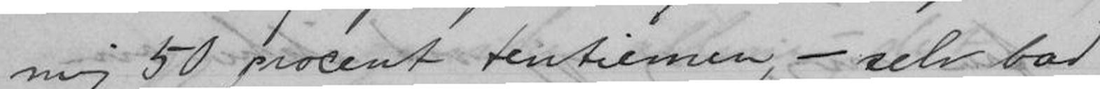mig 50 procent tentiemen, - selv bad
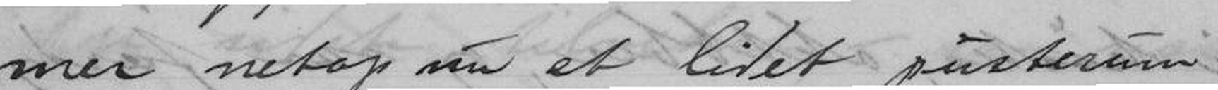mer, netop nu et lidet pusterum
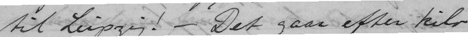til Leipzig! - Det gaar efter kilo
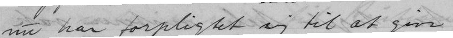nu har forpliktet sig til at give
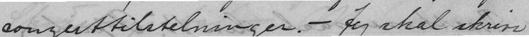conzerttilstelninger. - Jeg skal skrive
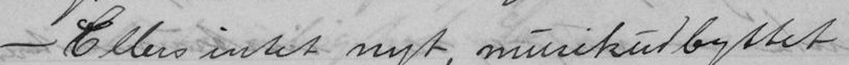- Ellers intet nyt, musikudbyttet
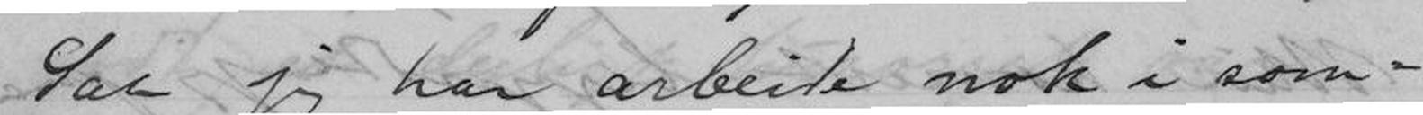Sa jeg har arbeide nok i som-
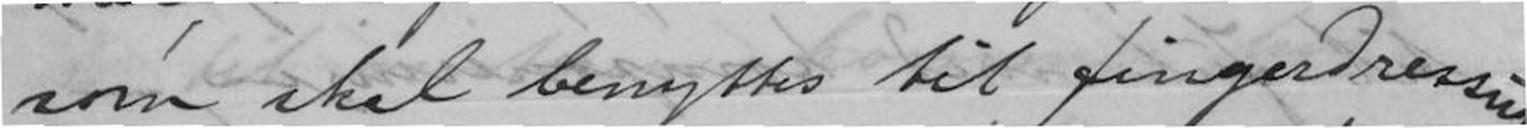som skal benyttes til fingerdressur,
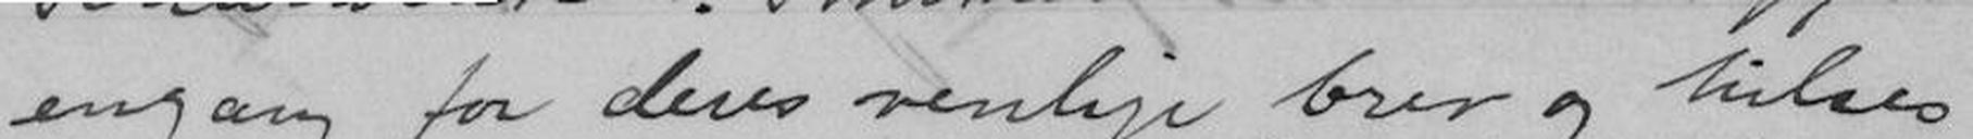engang for deres venlige brev og hilses
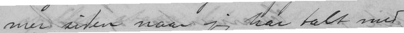mer siden naar jeg har talt med
